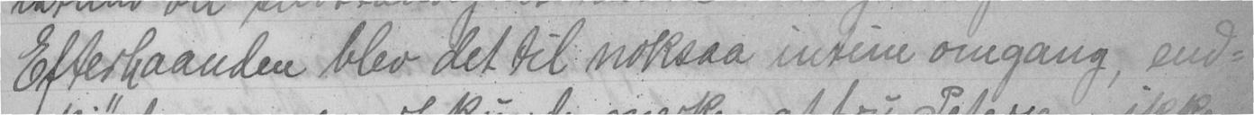Efterhaanden blev det til noksaa intim omgang, end-
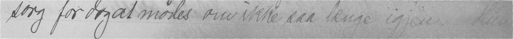sorg for dog at mødes om ikke saa lenge igjen.  Hun
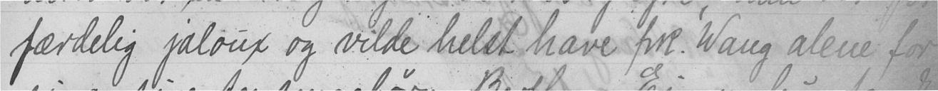færdelig jalous og vilde helst have frk. Wang alene for
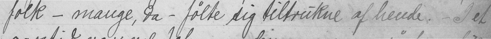folk - mange, da - følte sig tiltrukne af hende. - I et
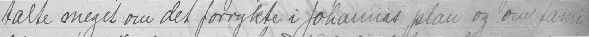talte meget om det forrykte i Johannas plan og om fami-
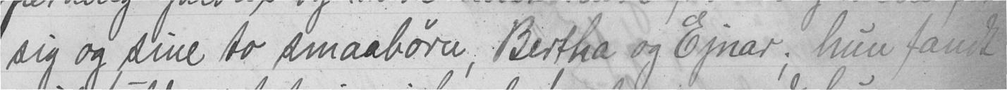sig og sine to smaabørn, Bertha og Ejnar, hun fandt
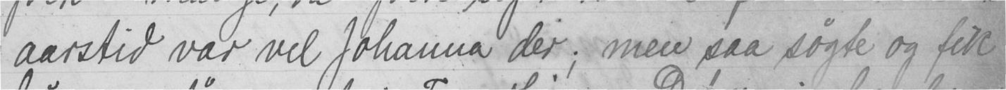aarstid var vel Johanna der; men saa søgte og fik
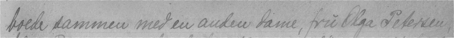boede sammen med en anden dame, fru Olga Petersen,
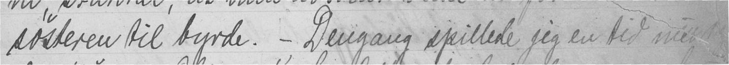søsteren til byrde. - Dengang spillede jeg en tid nu
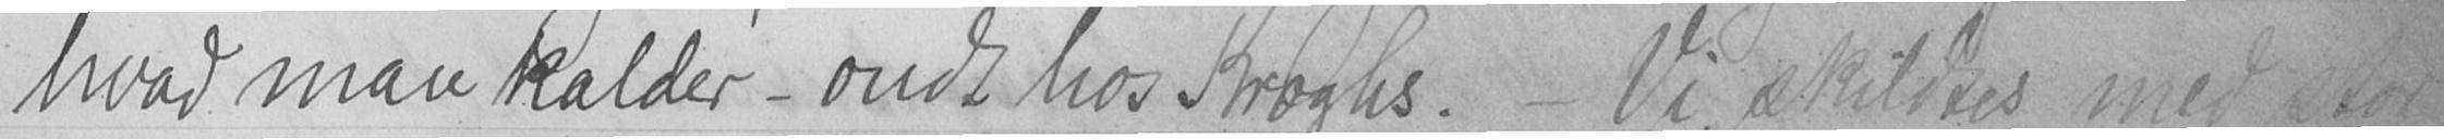hvad man kalder - ondt hos Kroghs. - Vi skildtes med stor
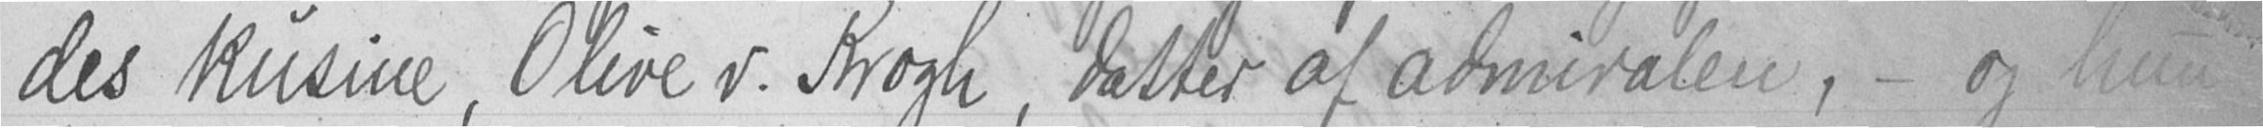des kusine, Olive v. Krogh, datter af admiralen, - og hun
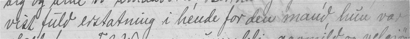vist fuld erstatning i hende for den mand, hun var
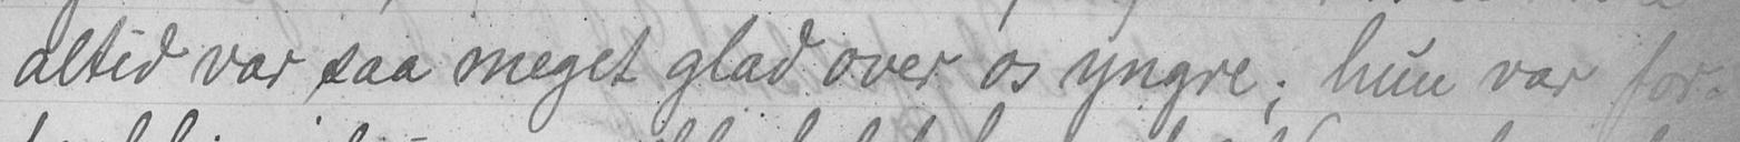altid var saa meget glad over os yngre; hun var for-
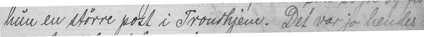hun en større post i Trondhjem. Det var jo hendes
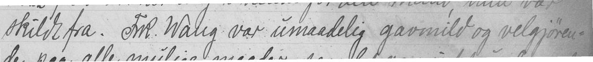skildt fra. Frk. Wang var umaadelig gavmild og velgjøren-
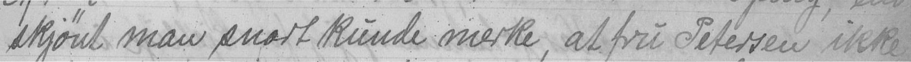skjønt man snart kunde merke, at fru Petersen ikke
